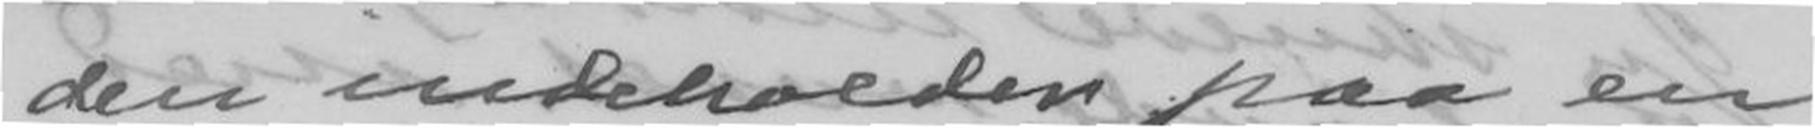den indeholder paa en
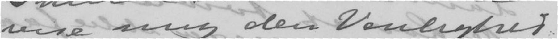vise mig den Venlighed
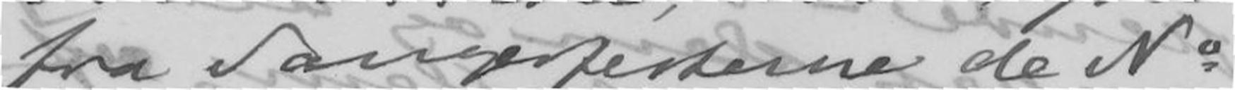fra Sangerfesterne de No
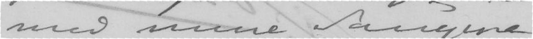med mine Sangere
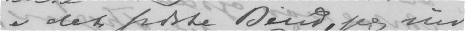i det sidste Bind, jeg nu
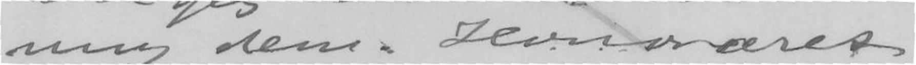mig dem. Honoraret
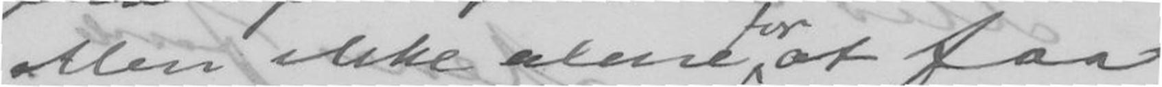Men ikke alene for at faa
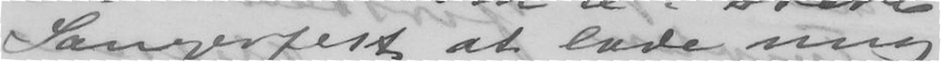Sangerfest at lade mig
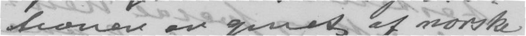tioner er givet af norske
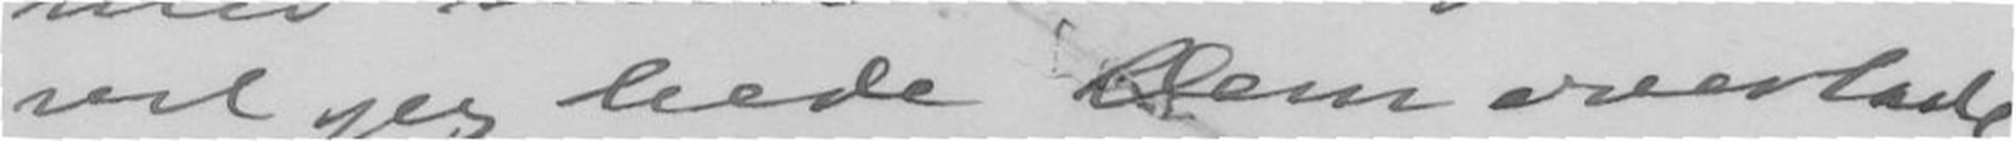vil jeg bee Dem overlade
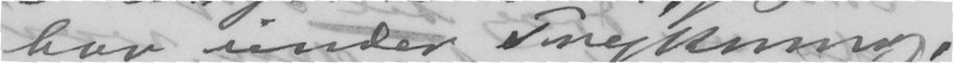har under Trykning;
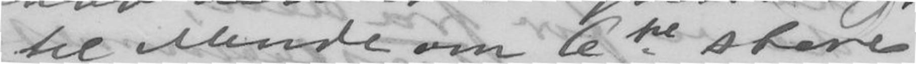til Minde om 6te Store
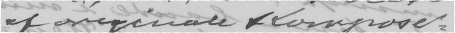af originale Kompose-
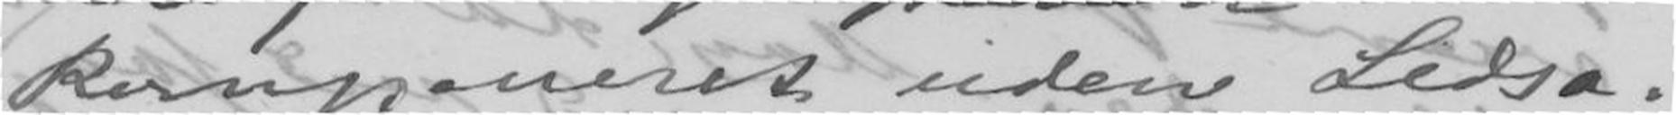komponeret uden Ledsa-
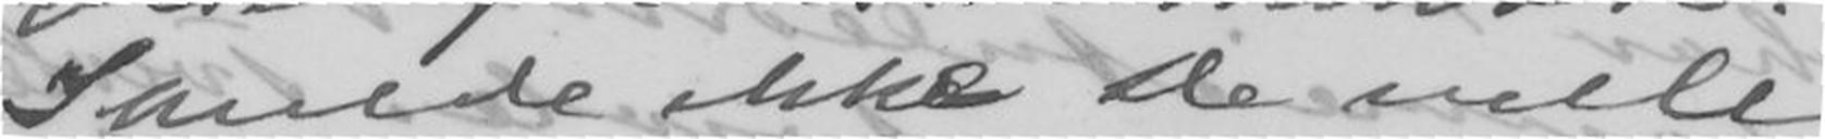Skulde ikke De ville
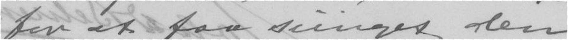for at faa sunget den
